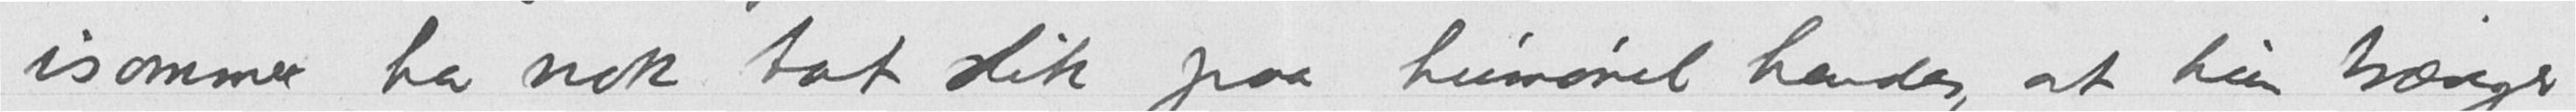isommer har nok tat slik paa humøret hendes at hun trænger
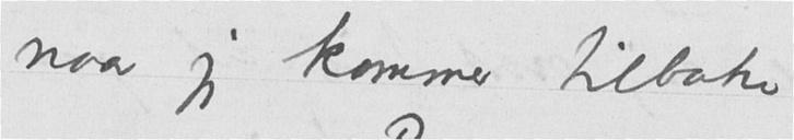naar jeg kommer tilbake
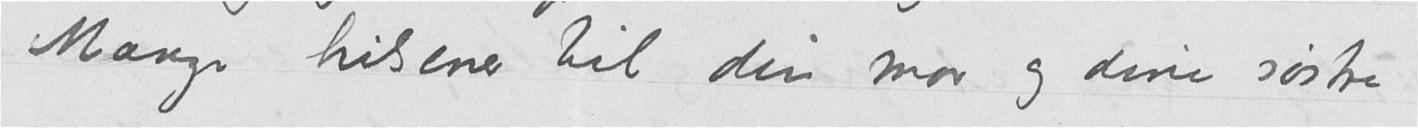Mange hilsener til din mor og dine søstre
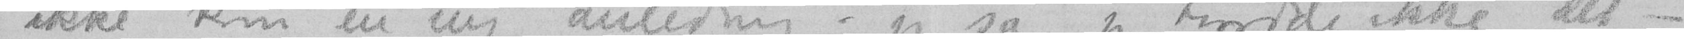ikke kom en ny anledning; jeg sa, jeg trodde ikke det -
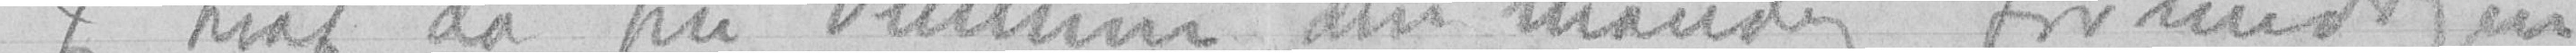Jeg traf da fru Vullum den mandag formiddagen
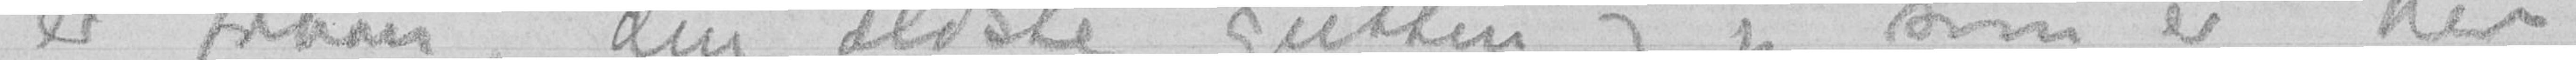er Johan, den ældste gutten og jeg som er her
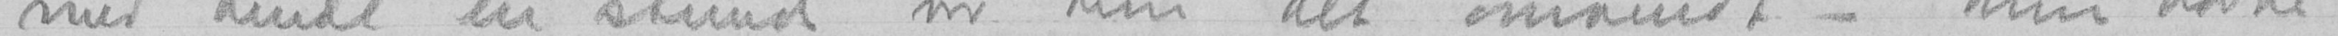med hende en stund var hun alt omvændt
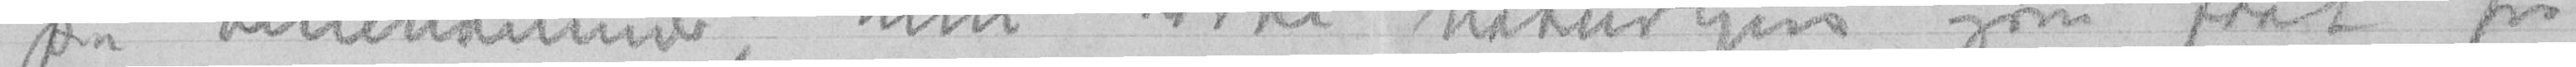paa Lillehammer; hun hadde naturligvis ogsaa faat for
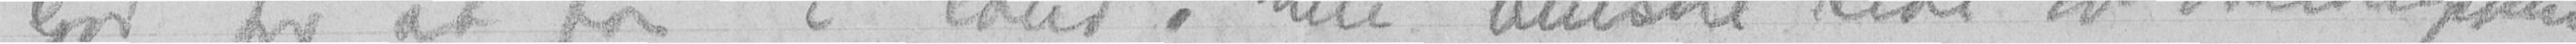god for at faa i land; hele venstre side av overkroppen
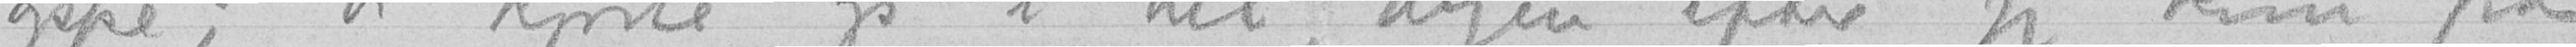oppe; vi kjørte op i bil, dagen efter jeg kom fra
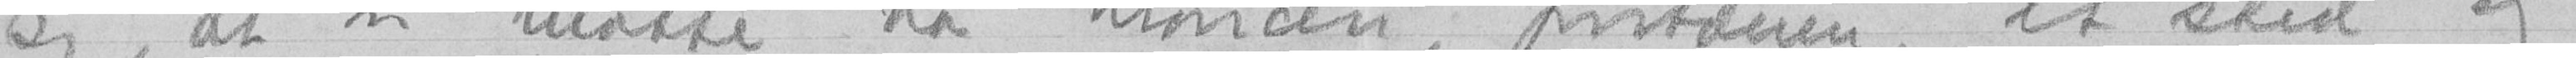sig, at vi måtte ha broncen, fontænen,et sted og
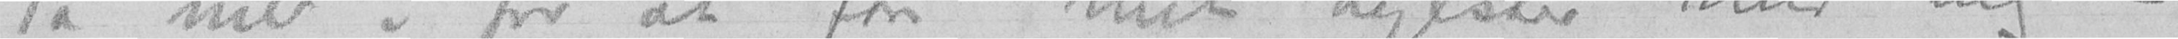ta mer i for at føre mit hylster med mig -
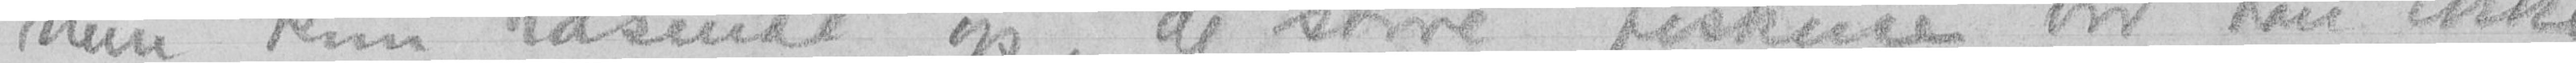men kom rasende op, de store fiskene var han ikke
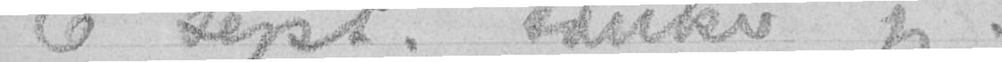6. Sept. tænker jeg.
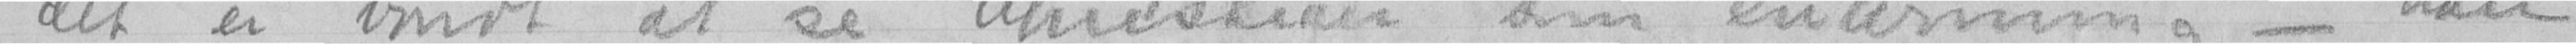det er vondt at se Christian som enarming - han
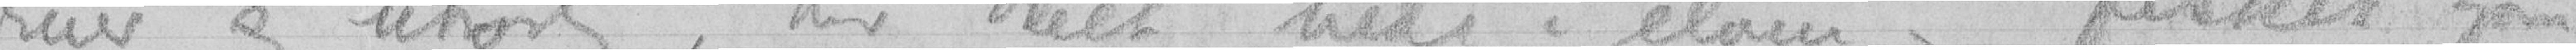greier seg utrolig, har været nede i elven og fisket ogsaa,
